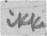ikke
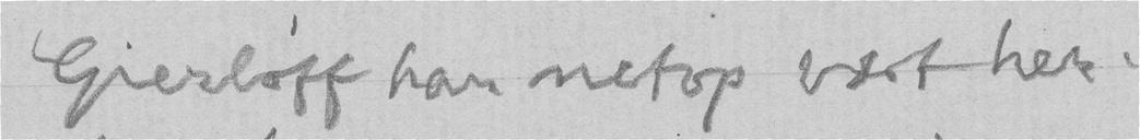Gierløff har netop vært her.
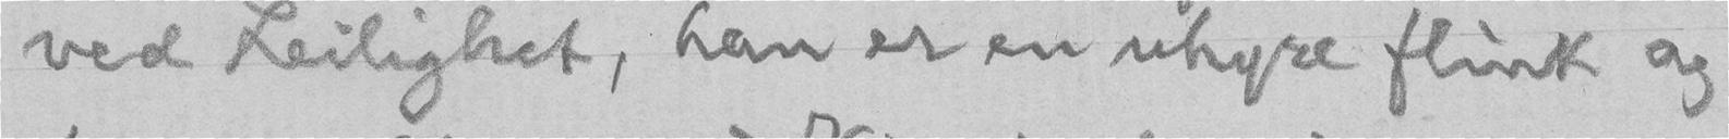ved Leilighet, han er en uhyre flink og
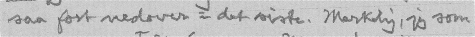saa fort nedover i det siste. Merkelig, jeg som
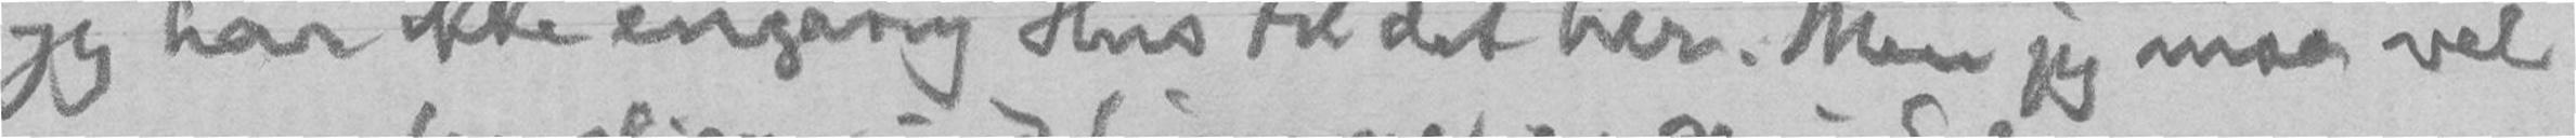jeg har ikke engang Hus til det her. Men jeg maa vel
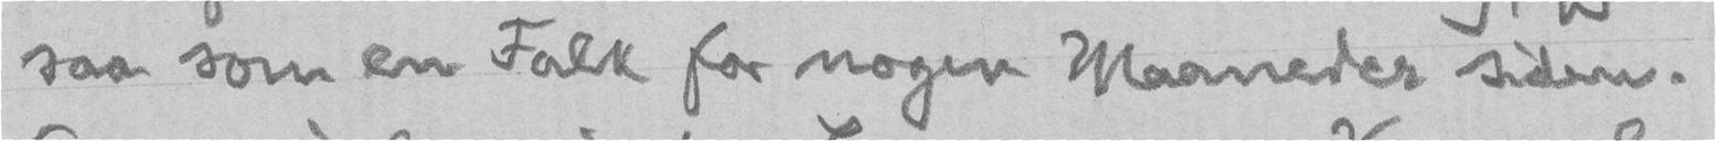saa som en Falk for nogen Maaneder siden.
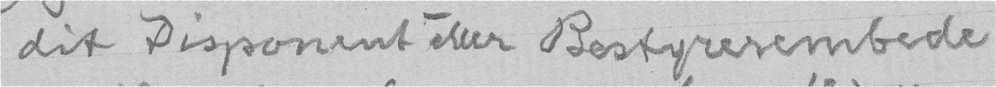dit Disponent eller Bestyrerembede
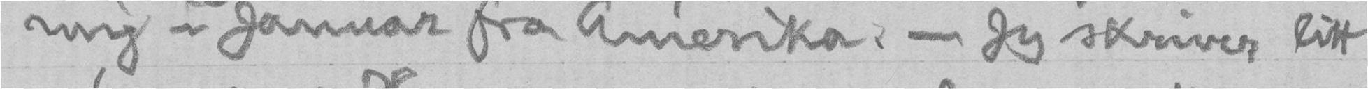mig i Januar fra Amerika. - Jeg skriver litt
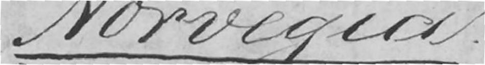Norvegia.
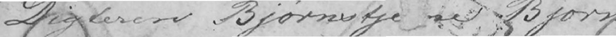Digteren Bjørnstjene Bjørnson
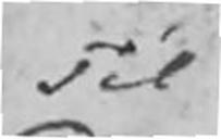Til
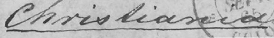Christiania
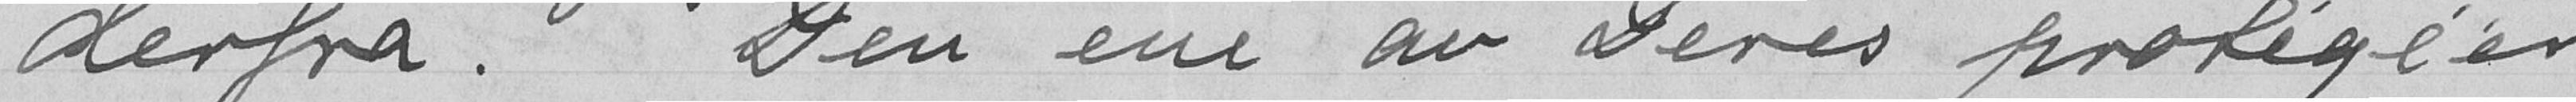derfra. Den ene av Deres protegeer
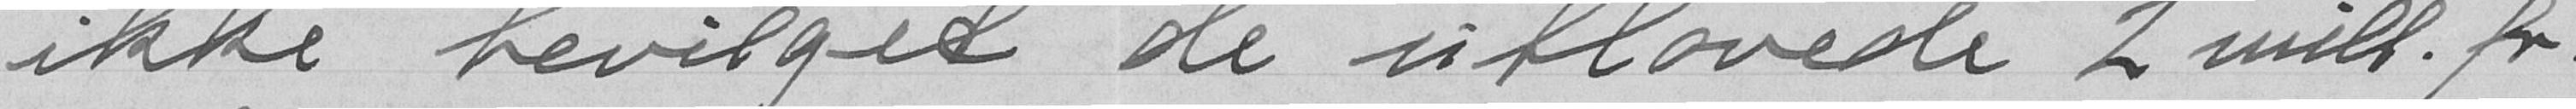ikke bevilget de utlovede 2 mill. fr.
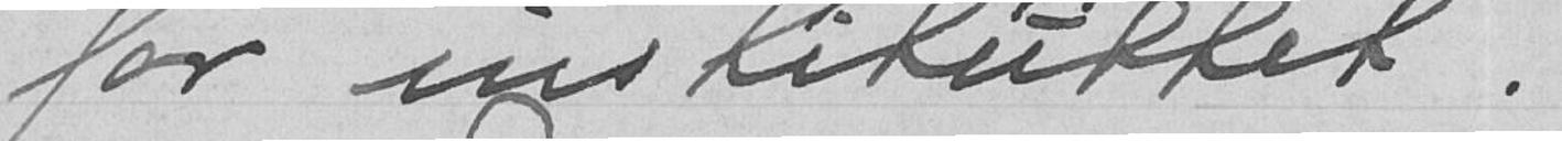for instituttet.
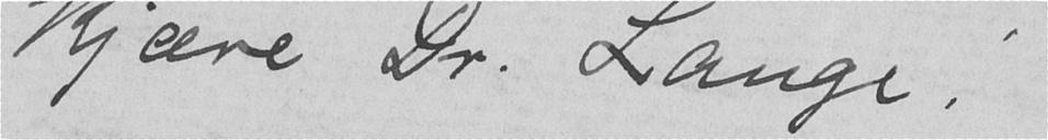Kjære Dr. Lange!
